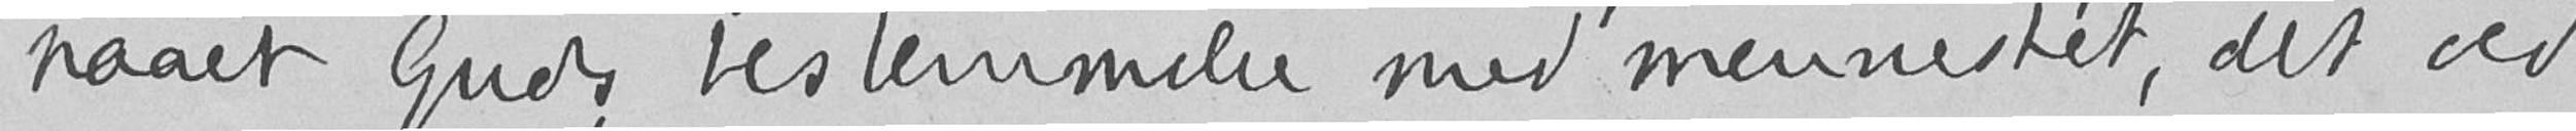naaet Guds bestemmelse med mennesket, det ved
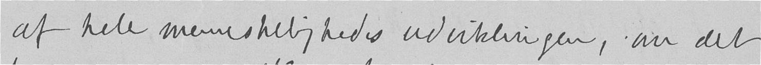af hele menneskelighedsudviklingen, om det
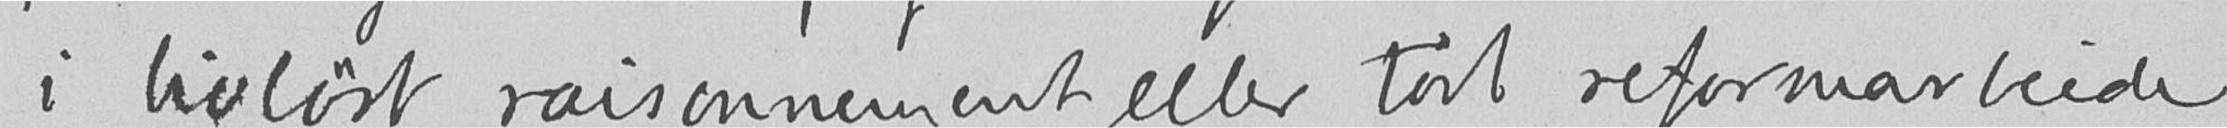i livløst raisonnement, eller tørt reformarbeide,
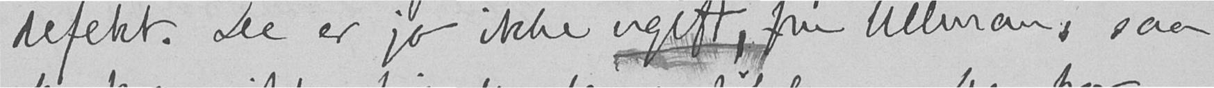defekt. De er jo ikke ugift, fru Ullman, saa
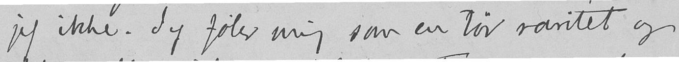jeg ikke. Jeg føler mig som en tør raritet og
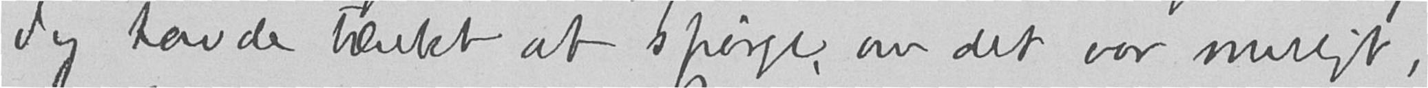Jeg havde tænkt at spørge, om det var muligt,
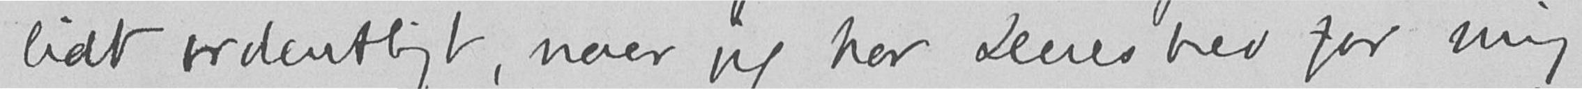lidt ordentligt, naar jeg har Deres brev for mig.
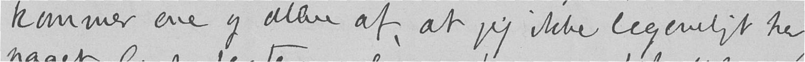kommer ene og alene af, at jeg ikke legemligt har
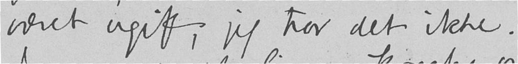været ugift, jeg tror det ikke.
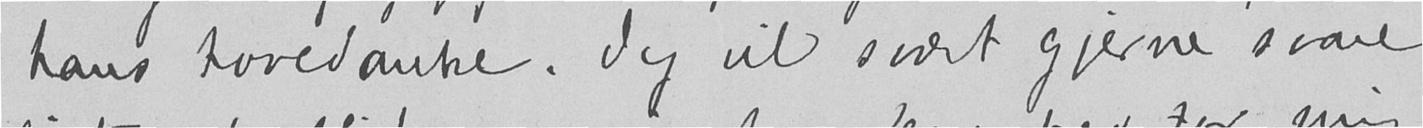hans hovedanke. Jeg vil svært gjerne svare
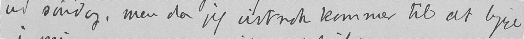ud søndag, men da jeg vistnok kommer til at ligge
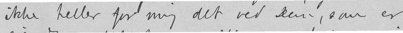ikke heller for mig det ved Dem, som er
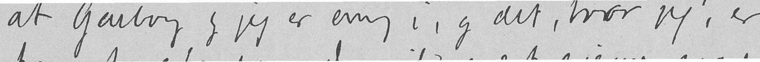at Garborg og jeg er enig i, og det, tror jeg, er
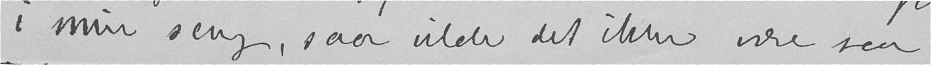i min seng, saa vilde det ikke være saa
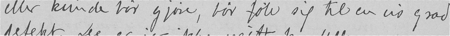eller kvinde bør gjøre, bør føle sig til en vis grad
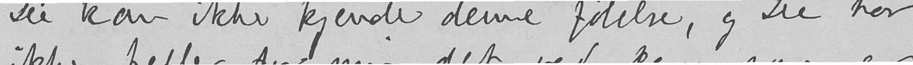De kan ikke kjende denne følelse, og De har
